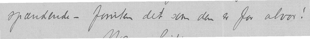spændende – foruten det som den er for alvor!
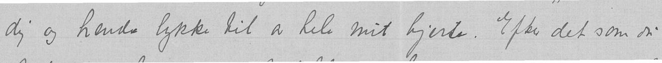dig og hende lykke til av hele mit hjerte. Efter det som du
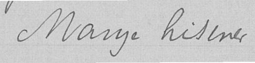Mange hilsener
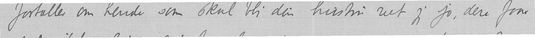fortæller om hende som skal bli din hustru vet jeg jo, dere faar
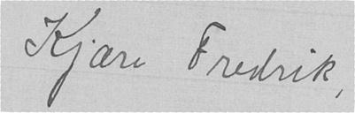Kjære Fredrik,
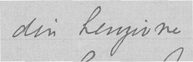din hengivne
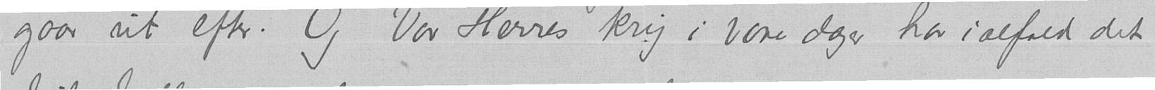gaar ut efter. Og Vor Herres krig i vore dager har ialfald det
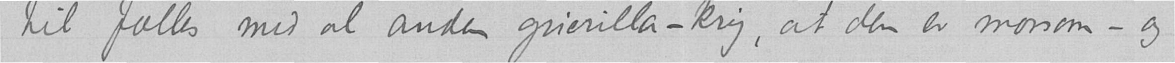til fælles med al anden guerilla-krig, at den er morsom – og
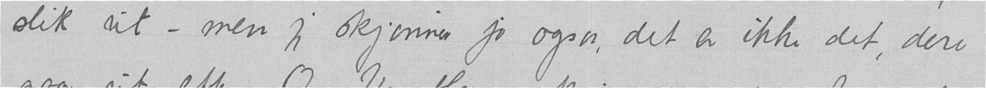slik ut – men jeg skjønner jo ogsaa, det er ikke det dere
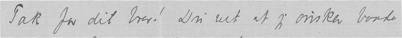Tak for dit brev! Du vet at jeg ønsker baade
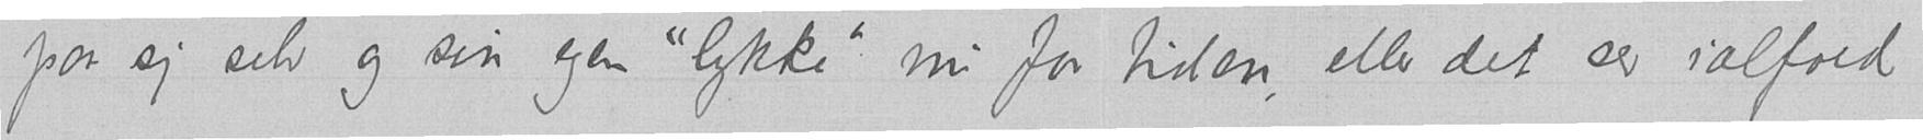paa sig selv og sin egen «lykke» nu for tiden, eller det ser ialfald
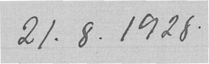21.8.1928.
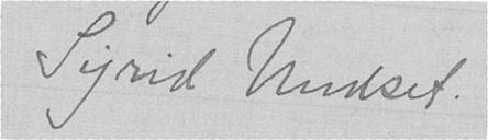Sigrid Undset.
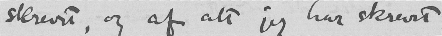skrevet, og af alt jeg har skrevet
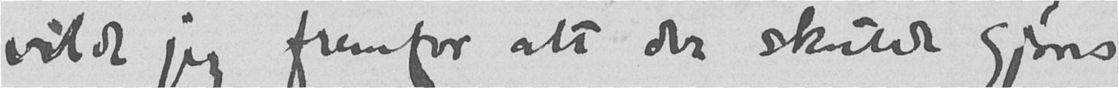vilde jeg fremfor alt der skulde gjøres
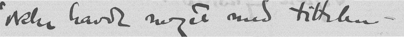ikke havde noget med tittelen -
